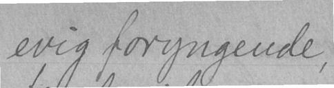evig foryngende,
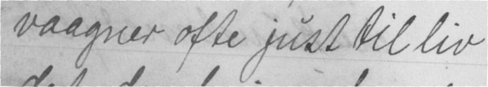vaagner ofte just til liv
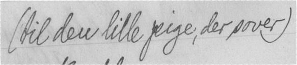(til den lille pige, der sover)
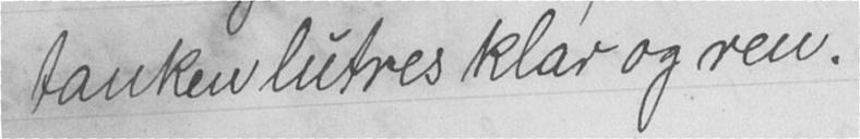tanken lutres klar og ren.
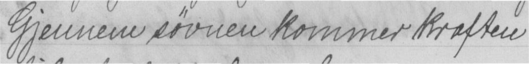Gjennem søvnen kommer kraften
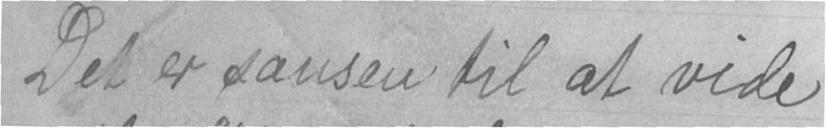Det er sansen til at vide
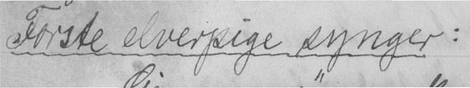Første elverpige synger:
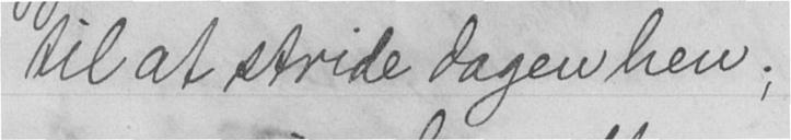til at stride dagen hen;
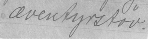æventyrstøv.
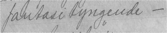fantasi tyngende -
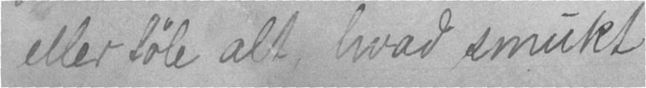eller føle alt, hvad smukt
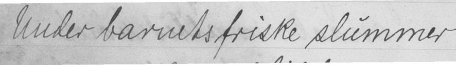Under barnets friske slummer
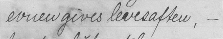evnen gives levesaften, -
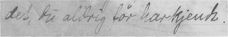det, du aldrig før har kjendt.
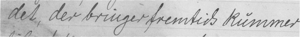det, der bringer fremtids kummer
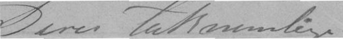Deres taknemlige
